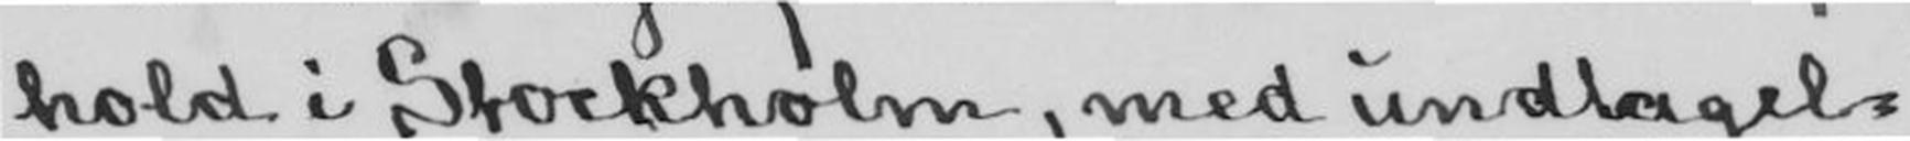hold i Stockholm, med undtagel-
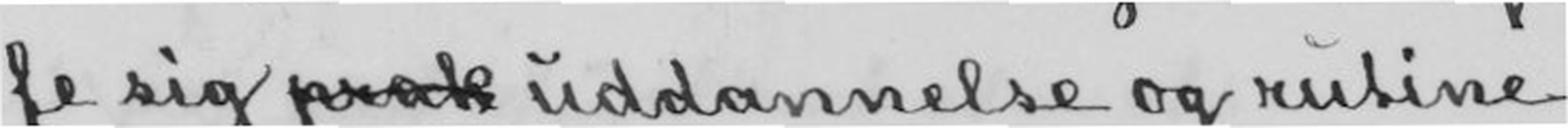fe sig prak uddannelse og rutine
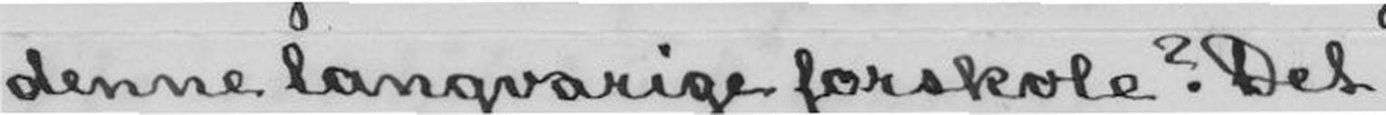denne langvarige forskole? Det
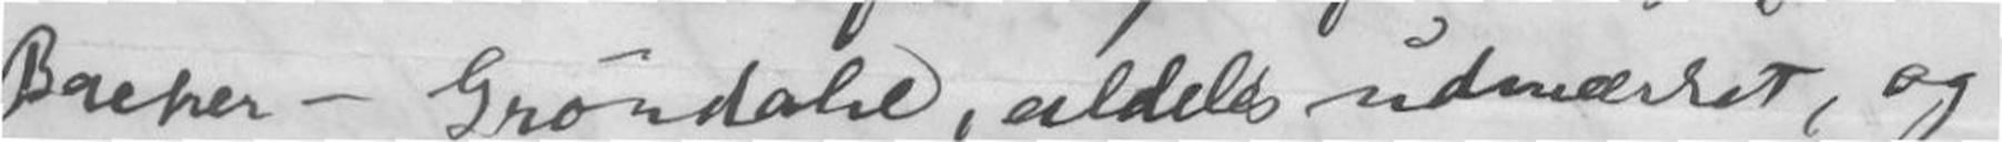Bacher-Grøndahl, aldeles udmærket, og
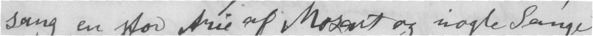sang en stor Arie af Mozart og nogle Sange
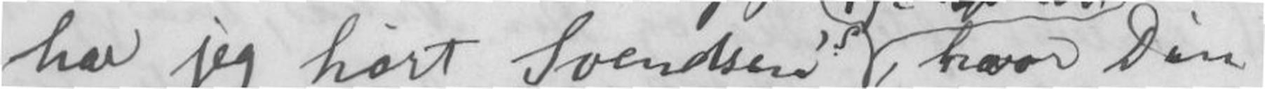har jeg hørt Svendsens , hvor Din
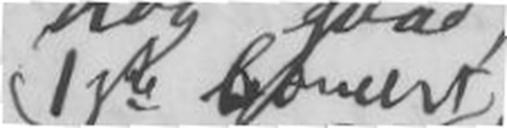1ste Concert
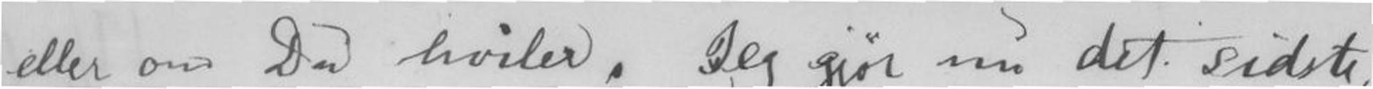eller om Du hviler. Jeg gjør nu det sidste,
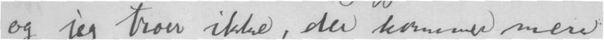og jeg troer ikke, der kommer mere
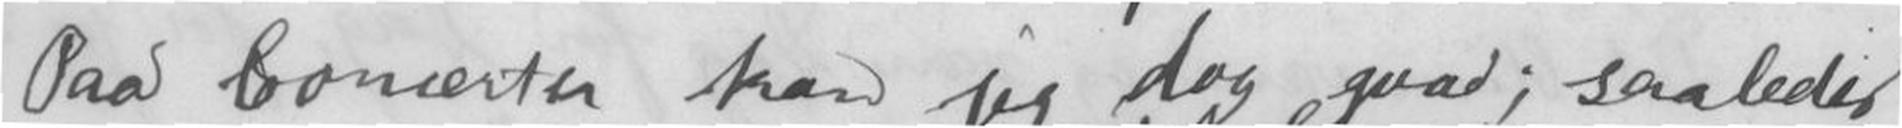Paa Concerter kan jeg dog gaa; saaledes
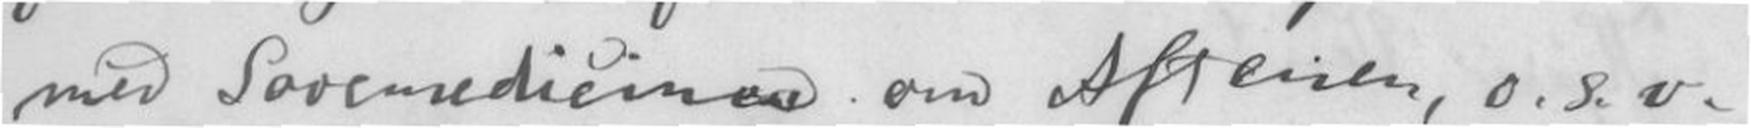med Sovemedicinen om Aftenen, o.s.v.
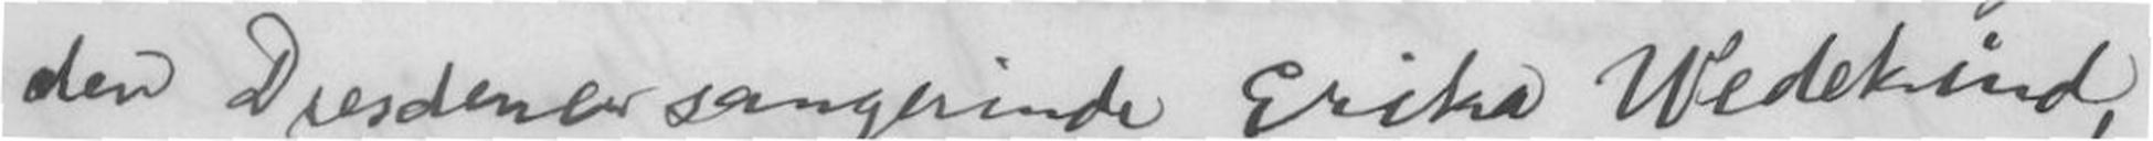den Dresdener sangerinde Erika Wedekind,
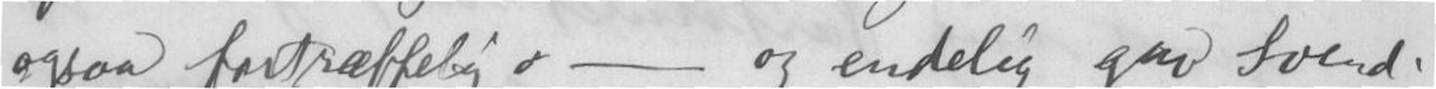ogsaa fortræffelig - og endelig gav Svend-
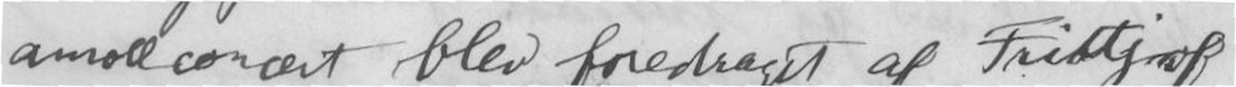amollconcert blev foredraget af Fridtjof
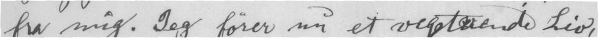fra mig. Jeg fører nu et vegeterende Liv,
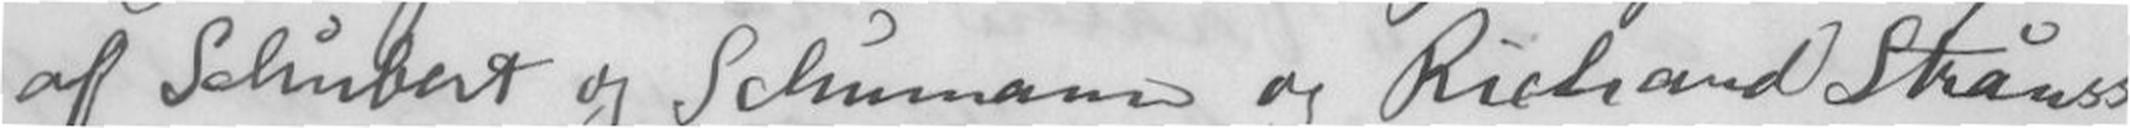af Schubert og Schuman og Richard Strauss,
